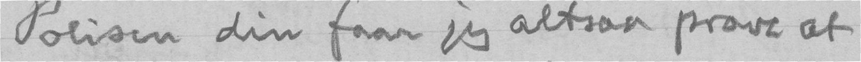Polisen din faar jeg altsaa prøve at
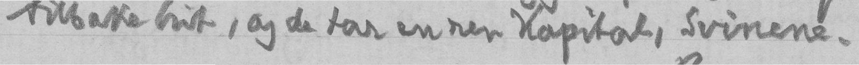tilbake hit, og de tar en ren Kapital, Svinene.
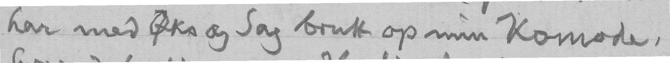har med Øks og Sag brutt op min Komode,
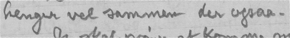henger vel sammen der ogsaa.
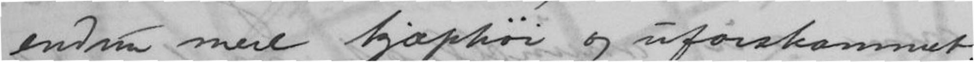endnu mer kjæphöi og uforskammet.
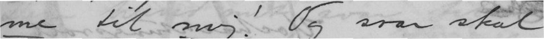me til mig! Og svar skal
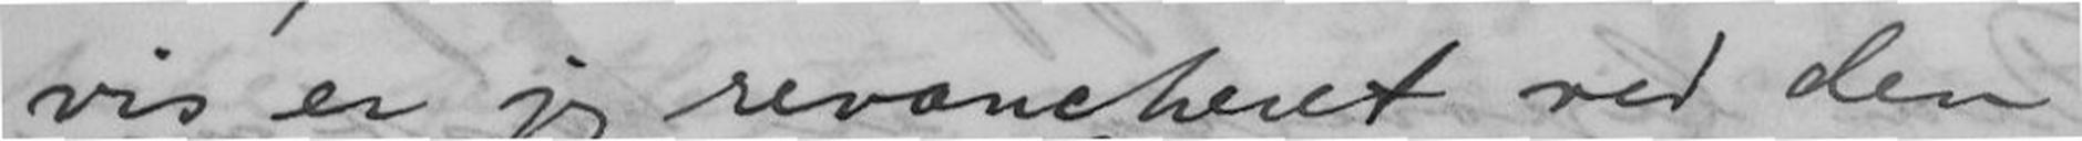vis er jeg revancheret ved den
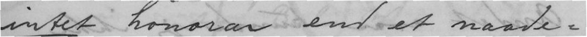intet honorar end et naade-
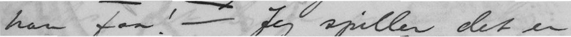han faa! - Jeg spiller, det er
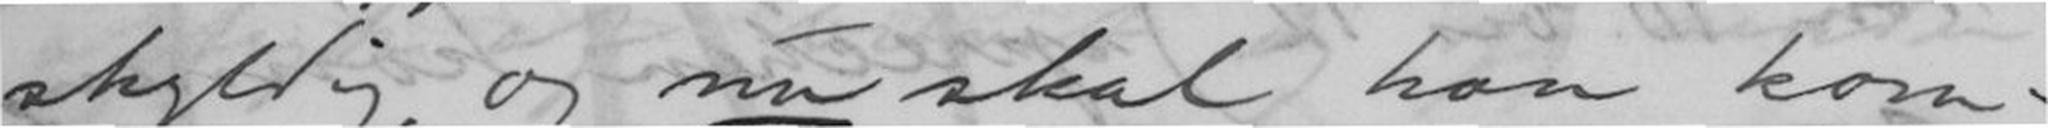skyldig, og nu skal han kom-
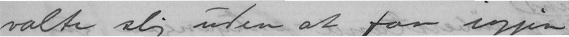valte slig uden at faa igjen
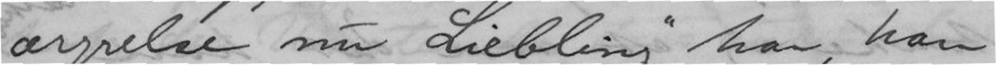ærgrelse nu "Liebling" har, han
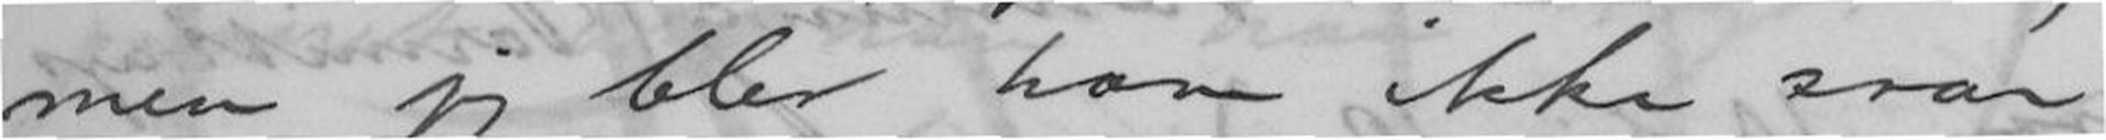men jeg blev ham ikke svar
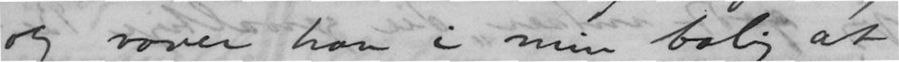og vover han i min bolig at
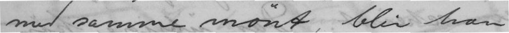med samme mönt, blir han
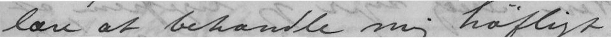lære at behandle mig höfligt,
